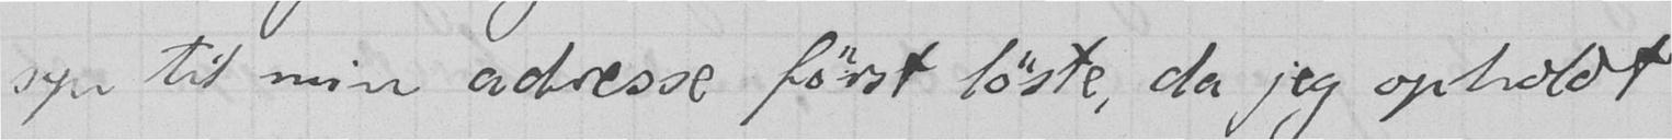syn til min adresse først løste, da jeg opholdt
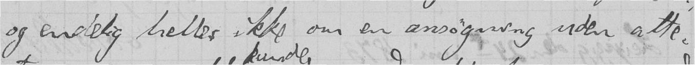og endelig heller ikke om en ansøgning uden atte-
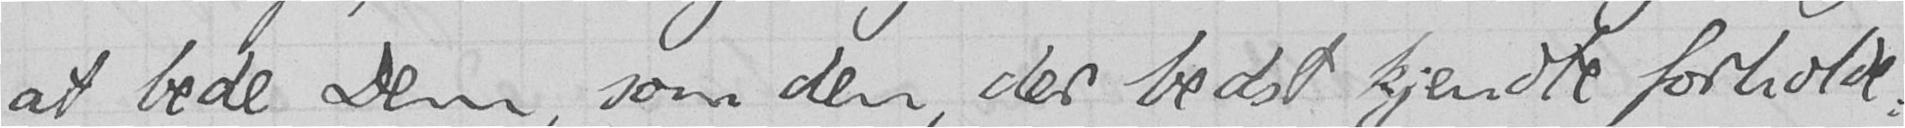at bede Dem, som den, der bedst kjendte forholde-
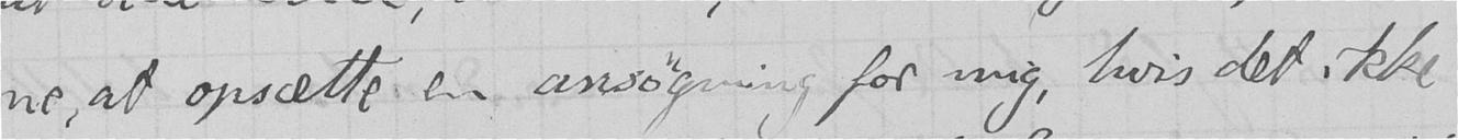ne, at opsætte en ansøgning for mig, hvis det ikke
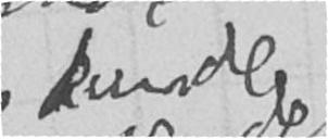kunde
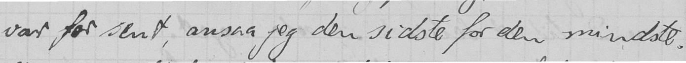var for sent, ansaa jeg den sidste for den mindste.
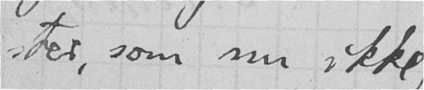ster, som nu ikke
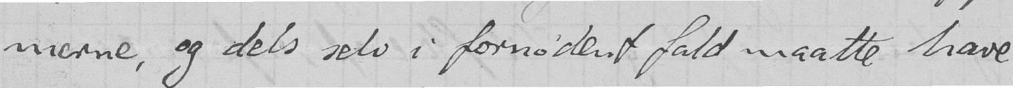merne, og dels selv i fornødent fald maatte have
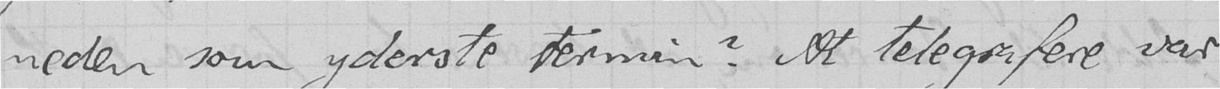neden som yderste termin? At telegrafere var
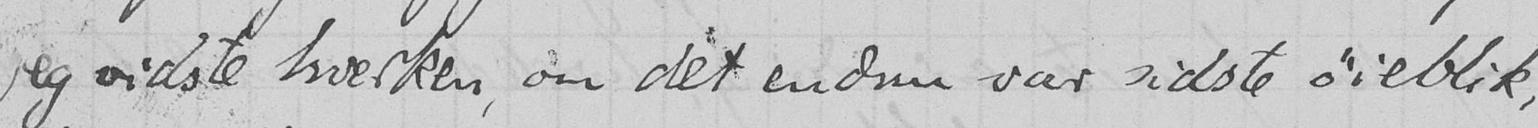jeg vidste hverken, om det endnu var sidste øieblik,
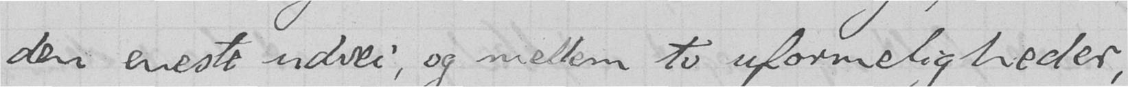den eneste udvei, og mellem to uformeligheder,
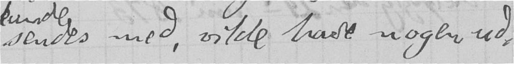sendes med, vilde have nogen ud-
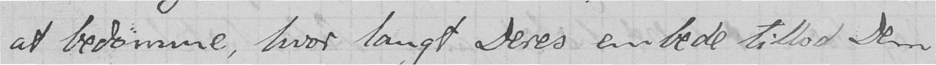at bedømme, hvor langt Deres embede tillod Dem
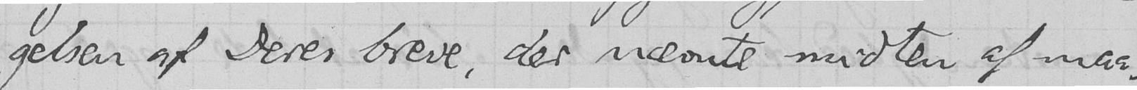gelsen af Deres breve, der nævnte midten af maa-
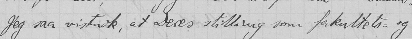Jeg saa vistnok, at Deres stilling som fakultets- og
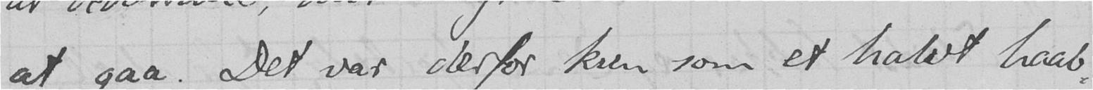at gaa. Det var derfor kun som et halvt haab-
